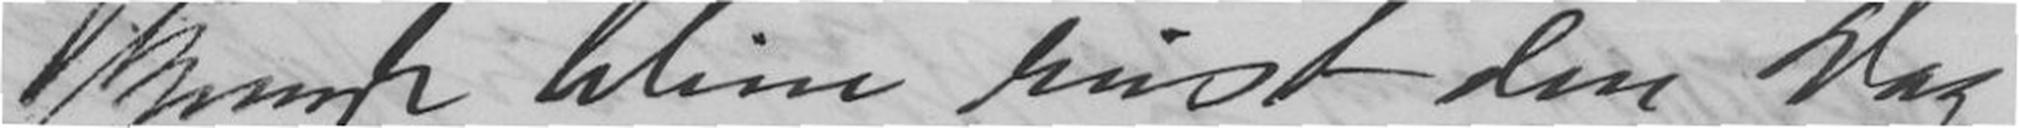kunde blive reist den Dag.
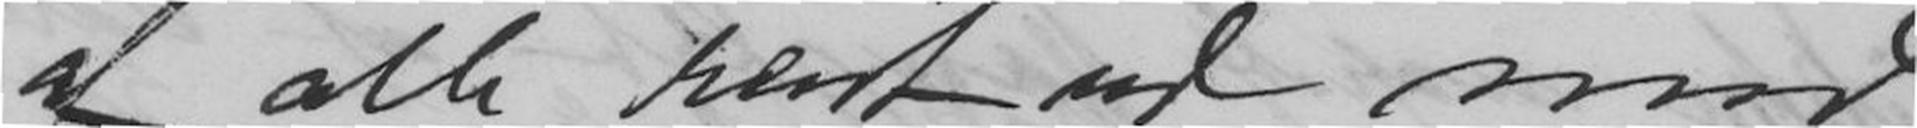af alle rent ud med
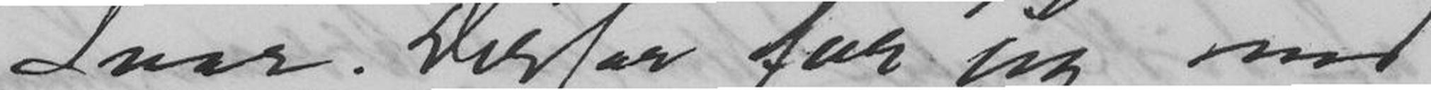Svar. Derfor for jeg ned
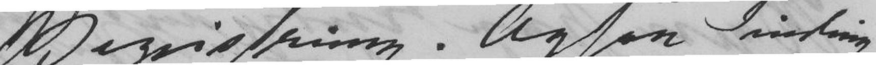Begeistring. Ogsaa Sinding
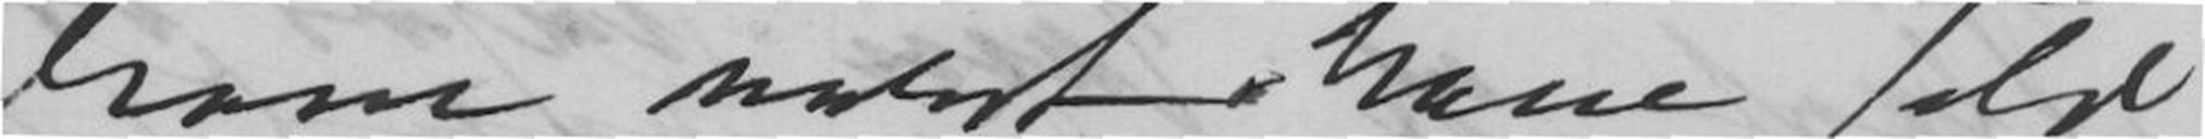ham været ham selv
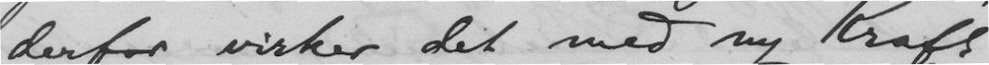derfor virker det med ny Kraft
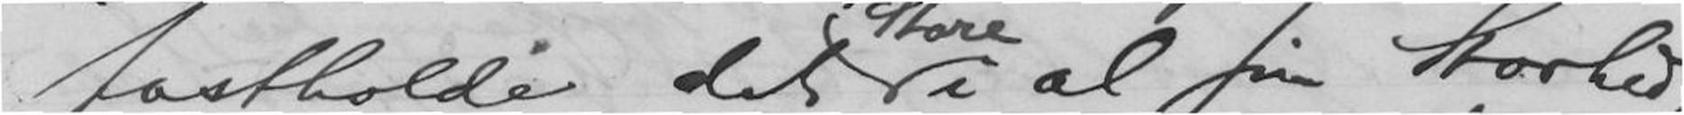fastholde det i al sin Storhed,
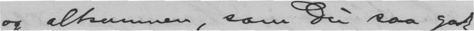og altsammen, som Du saa godt
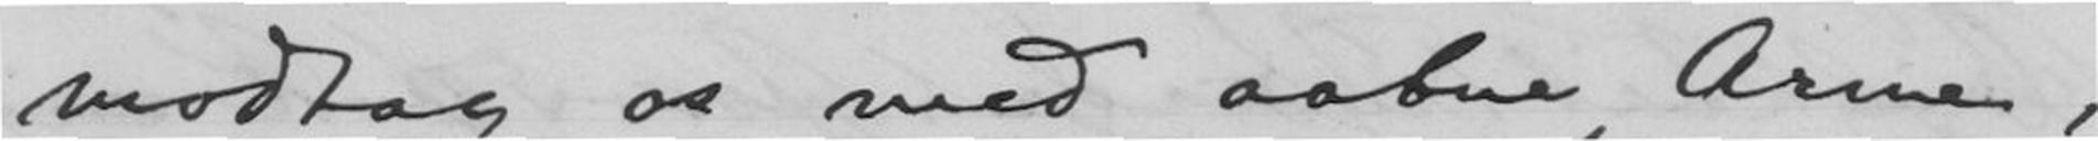modtog os med aabne Arme,
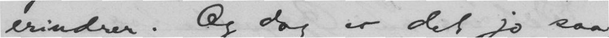erindrer. Og dog er det jo saa,
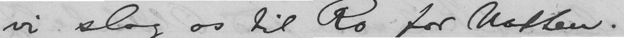vi slog os til Ro for Natten.
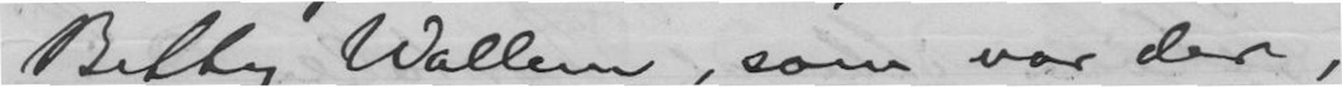Betty Wallem, som var der,
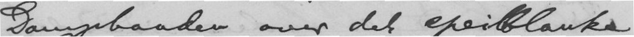Dampbaaden over det speilblanke
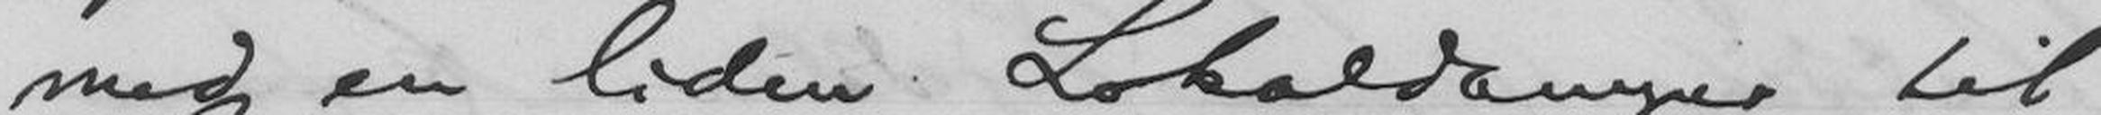med en liden Lokaldamper til
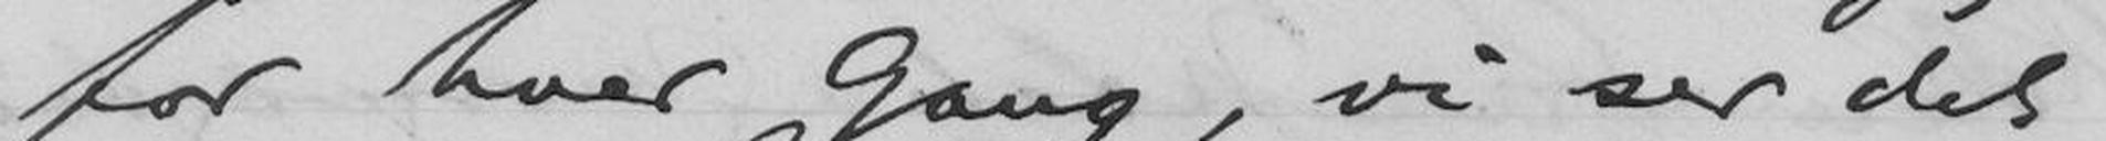for hver Gang, vi ser det
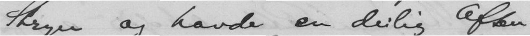Stryn og havde en deilig Aften-
